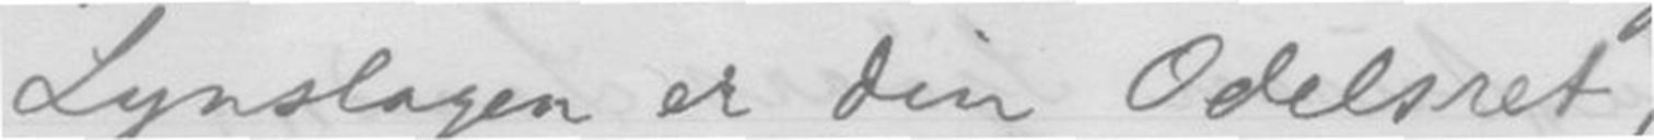Lynslagen er din Odelsret
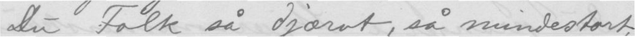Du Folk så djærvt, så mindestort,
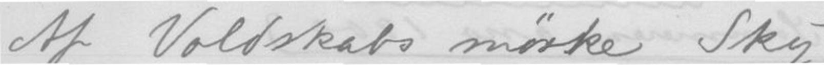Af Voldskabs mørke Sky
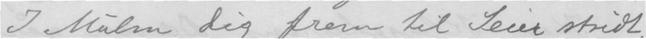I Mulm dig frem til Seier stridt
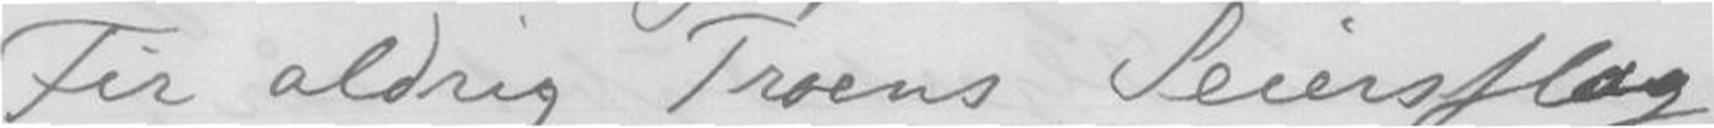Fir aldrig Troens Seiersflag
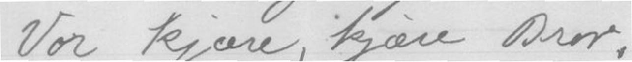Vor kjære, kjære Bror.
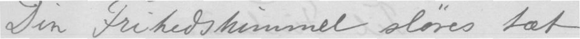Din Frihedshimmel sløres tæt
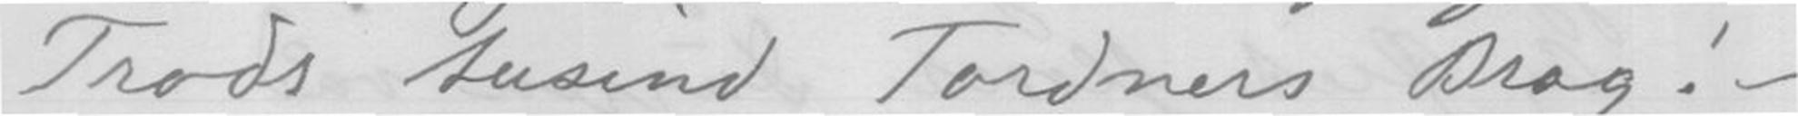Trods tusind Tordners Brag! -
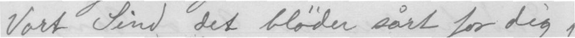Vort Sind det bløder sårt for dig,
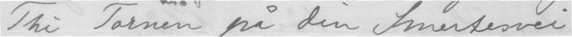Thi Tornen på din Smertesvei
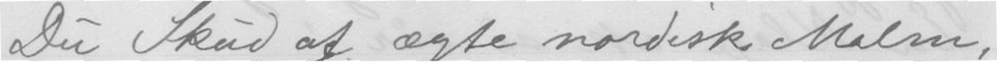Du Skrud af ægte nordisk Malm,
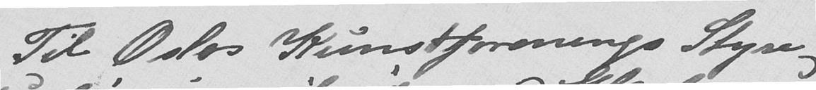Til Oslos Kunstforenings Styre
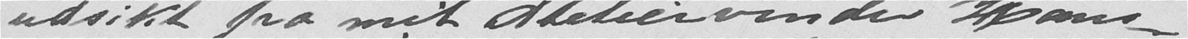utsikt fra mit Ateliervindu Hans-
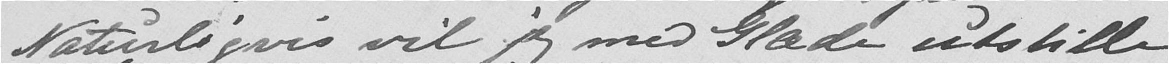Naturligvis vil jeg med Glæde utstille
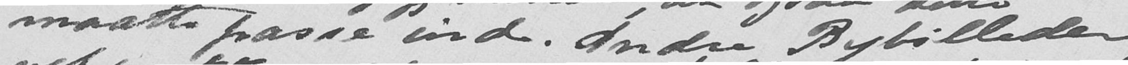maatte passe ind. Andre Bybilleder
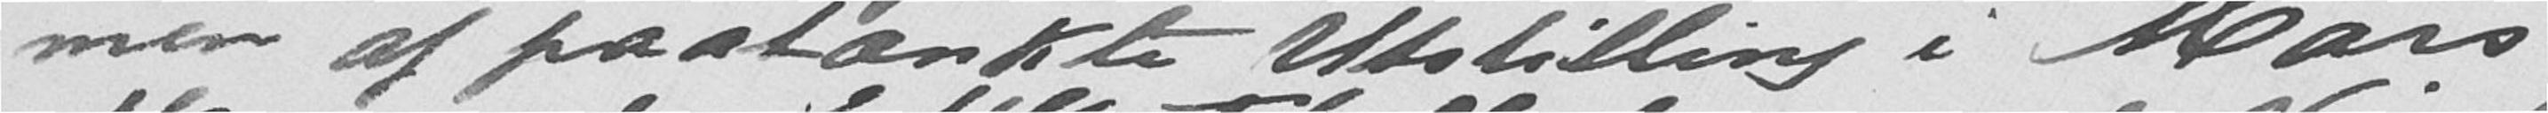men af paatænkte Utstilling i Mars
